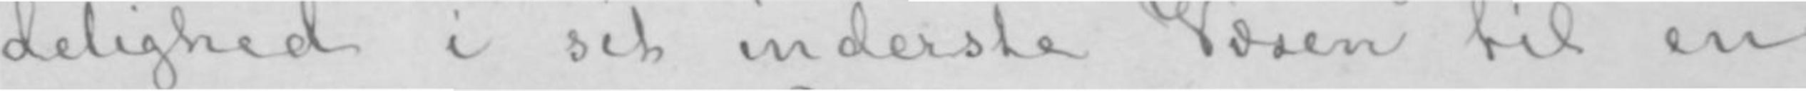delighed i sit inderste Væsen til en
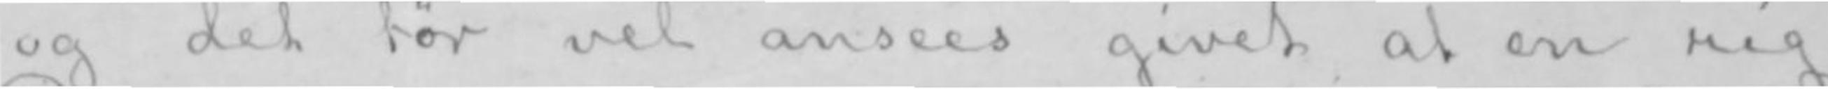og det tør vel ansees givet at en rig
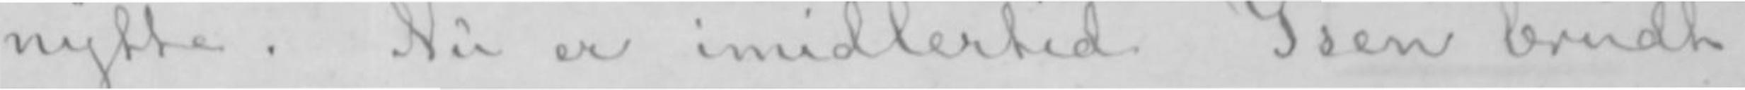nytte. Nu er imidlertid Isen brudt,
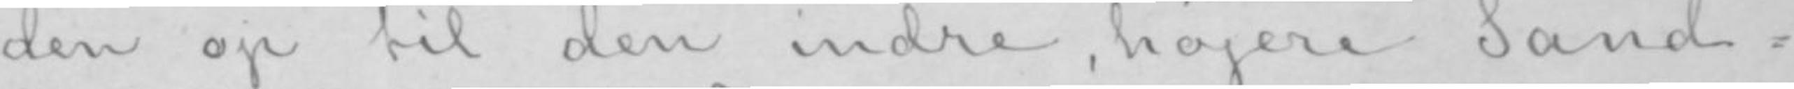den op til den indre, højere Sand-
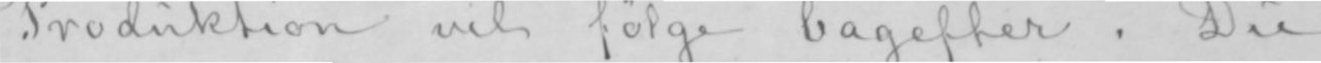Produktion vil følge bagefter. Du
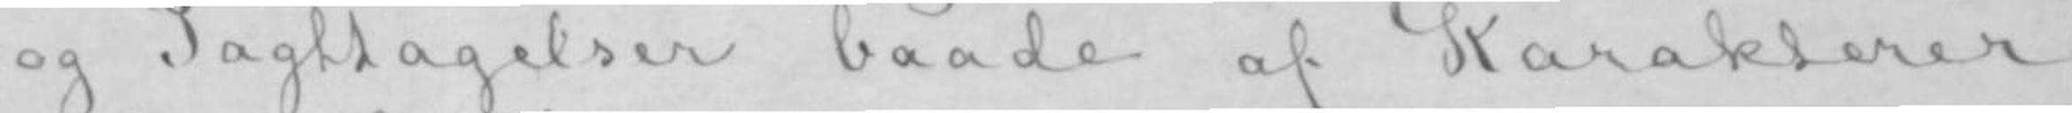og Iagttagelser baade af Karakterer
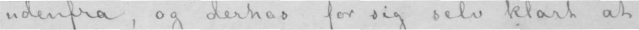udenfra, og derhos for sig selv klart at
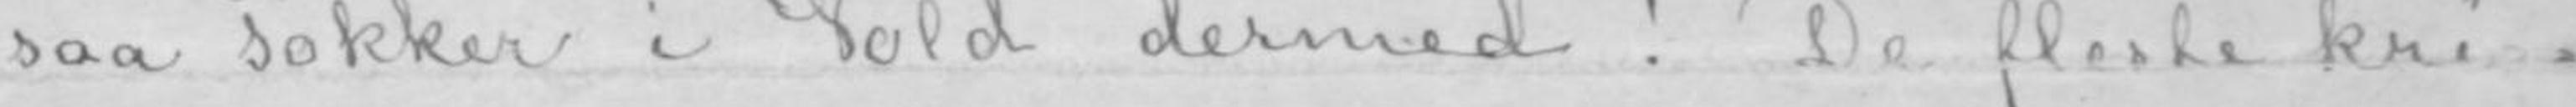saa Pokker i Vold dermed! De fleste kri-
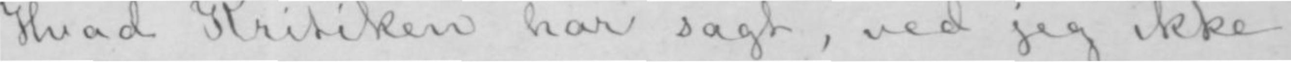Hvad Kritiken har sagt, ved jeg ikke;
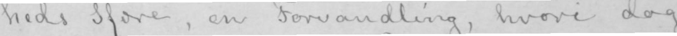heds Sfære, en Forvandling, hvori dog
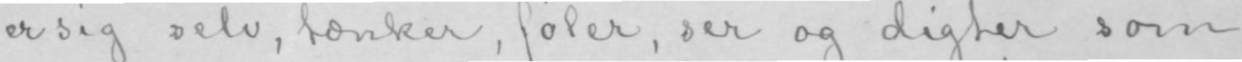er sig selv, tænker, føler, ser og digter som
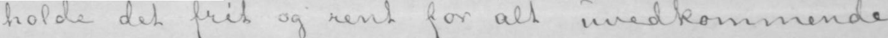holde det frit og rent for alt uvedkommende
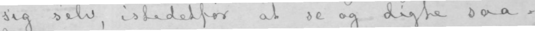sig selv, istedetfor at se og digte saa-
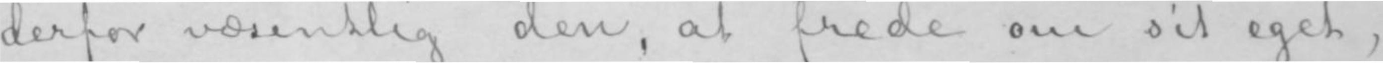derfor væsentlig den, at frede om sit eget,
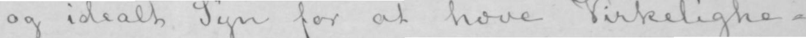og idealt Syn for at hæve Virkelighe-
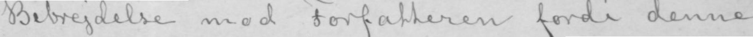Bebrejdelse mod Forfatteren fordi denne
